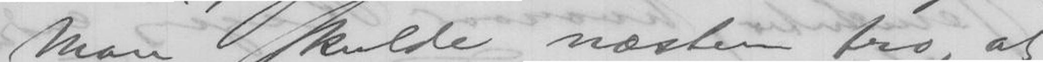Man skulde næsten tro, at
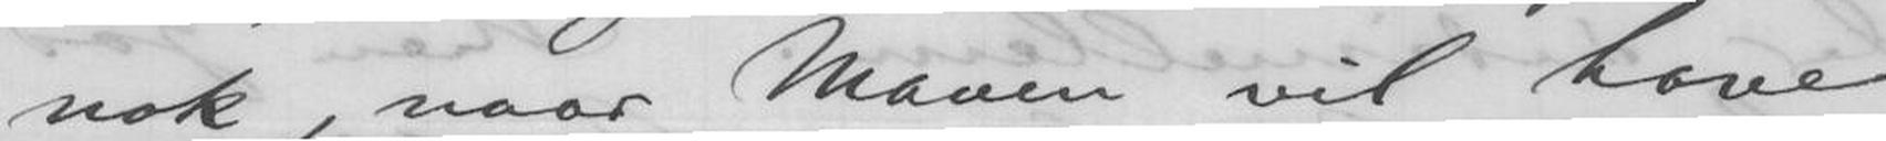nok, naar Maven vil have
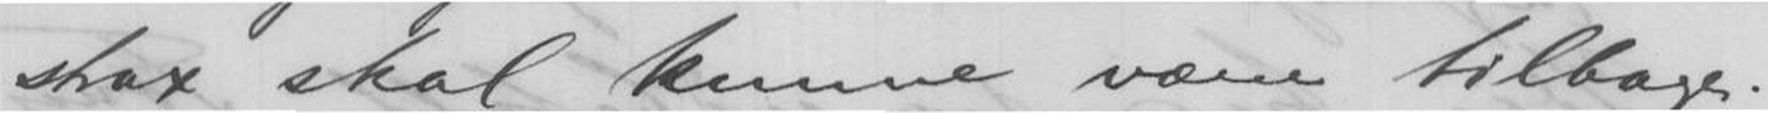strax skal kunne være tilbage.
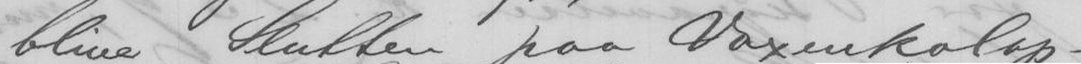blive Slutten paa Voxenkolop
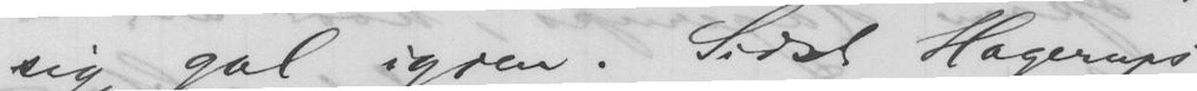sig gal igjen. Sidst Hagerups
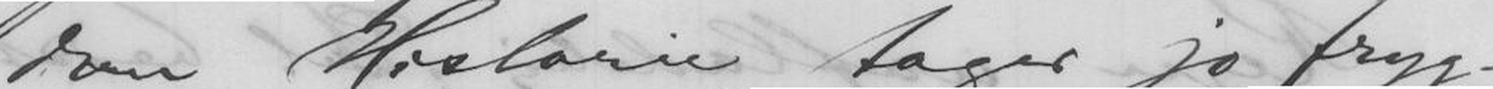dan Historie tager jo fryg
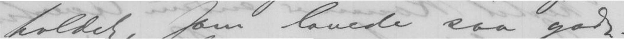holdet, som lovede saa godt.
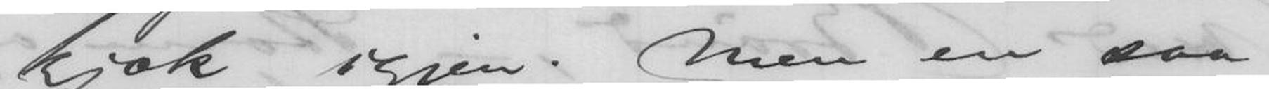kjæk igjen. Men en saa
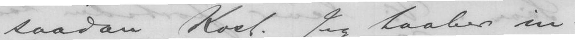saadan Kost. Jeg haaber in
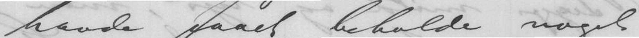havde faaet beholde noget
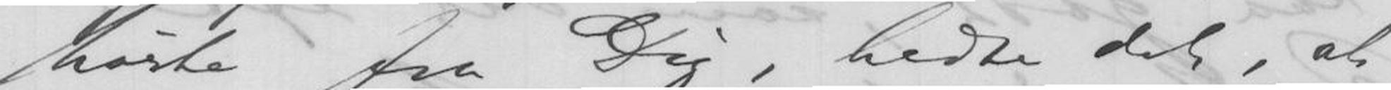hørte fra Dig, hedte det, at
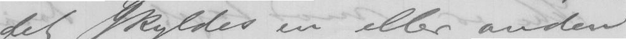det skyldes en eller anden
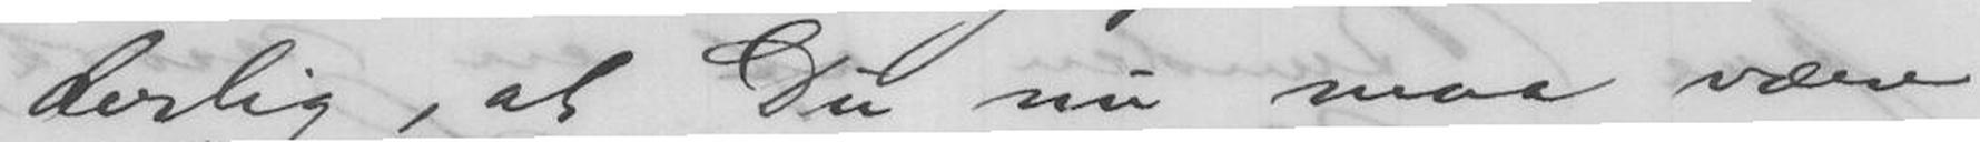derlig, at Du nu maa være
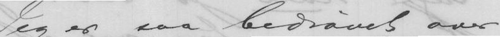Jeg er saa bedrøvet over
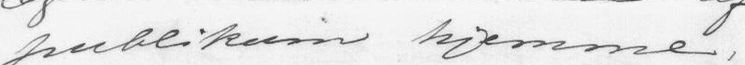publikum hjemme,
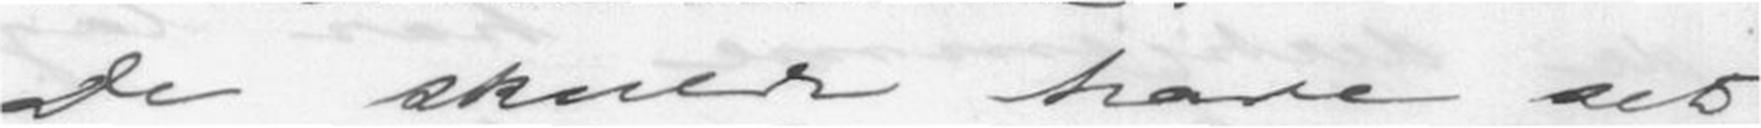De skulde have set
